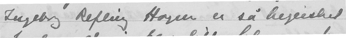Ingeborg Refling Hagen er så begeistret
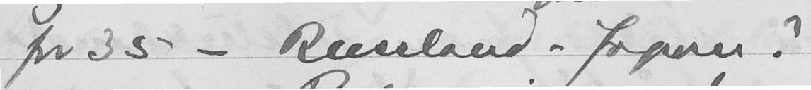for 35 - Russland - Japan?
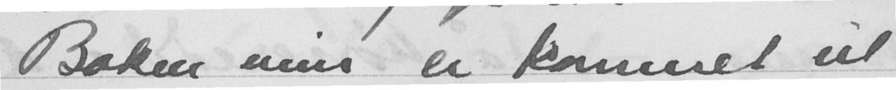Boken min er kommet ut
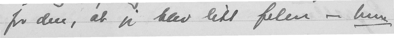for den, at jeg blev litt felen - hun
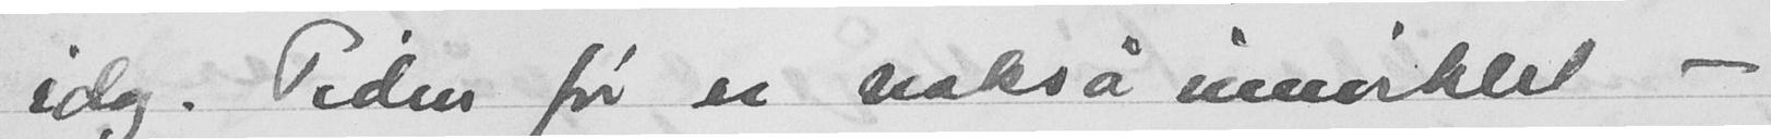idag. Tiden før er nokså innviklet -
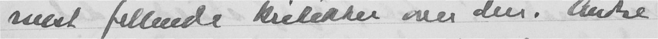mest fellende kritikker over den. Andre
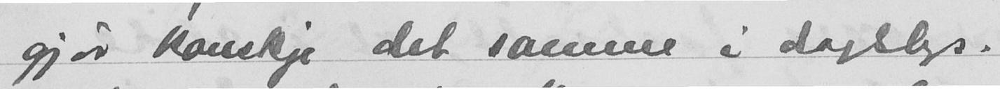gjør kanskje det samme i dagslys.
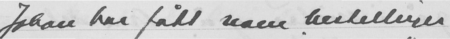Johan har fått noen bestellinger
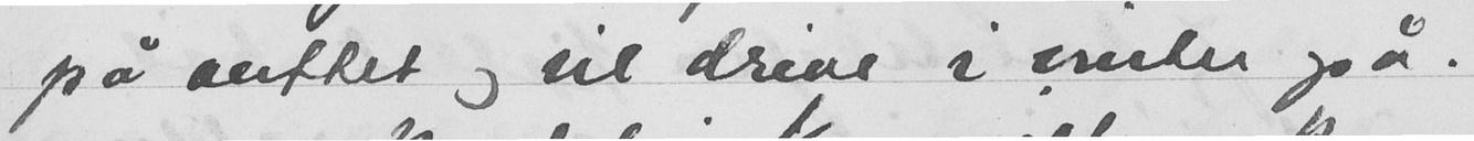på verftet og vil drive i vinter også!
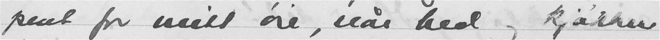pent for mitt øie, når bed og kjøkken
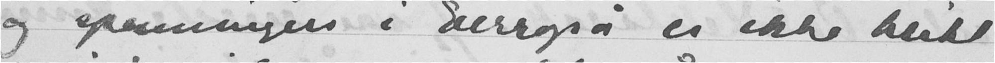og spenningen i Europa er ikke blitt
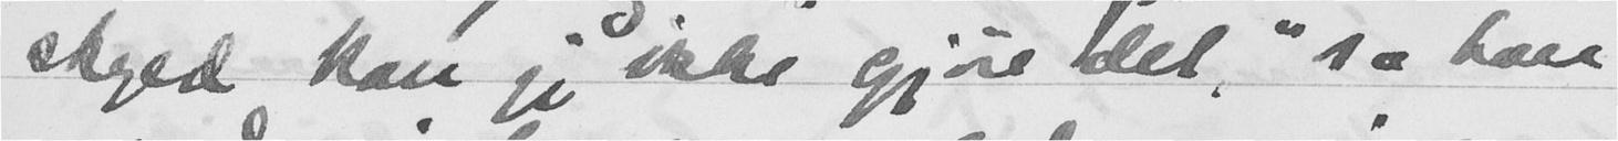skyld kan jeg ikke gjøre det," sa han
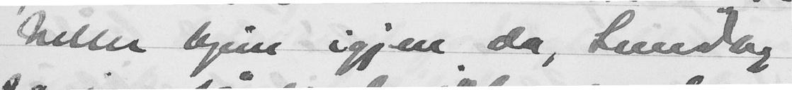heller hjem igjen da, Sundby
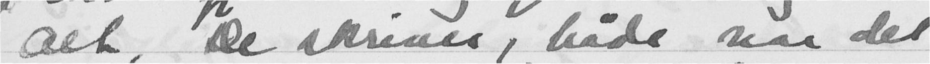alt, De skriver, både når det
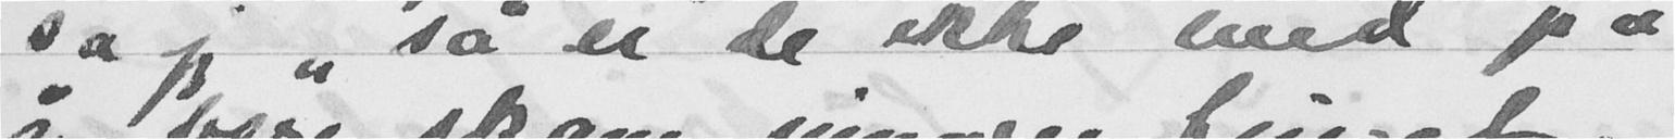sa jeg "så er de ikke med på
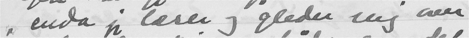"enda jeg læser og gleder mig over
